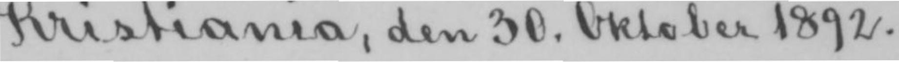Kristiania, den 30. Oktober 1892.
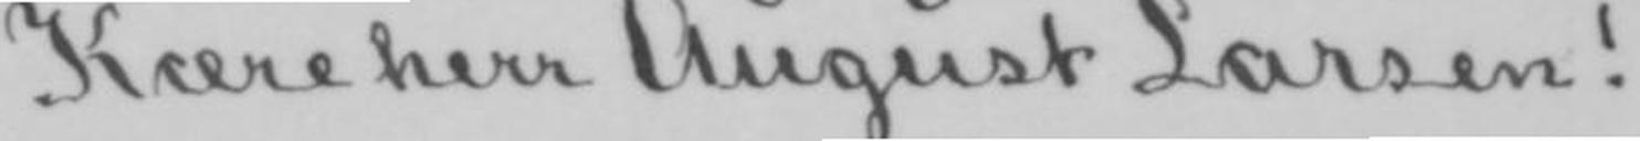Kære herr August Larsen!
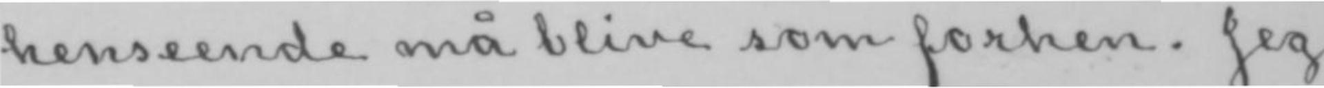henseende må blive som forhen. Jeg
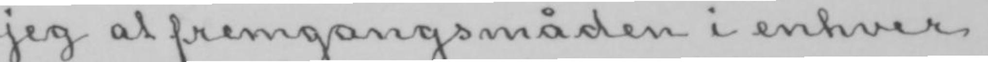jeg at fremgangsmåden i enhver
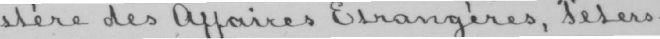stère des Affaires EtrangèresPeters-
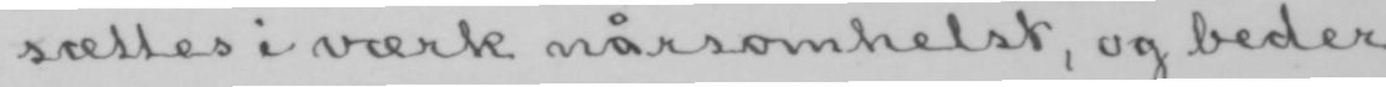sættes i værk nårsomhelst, og beder
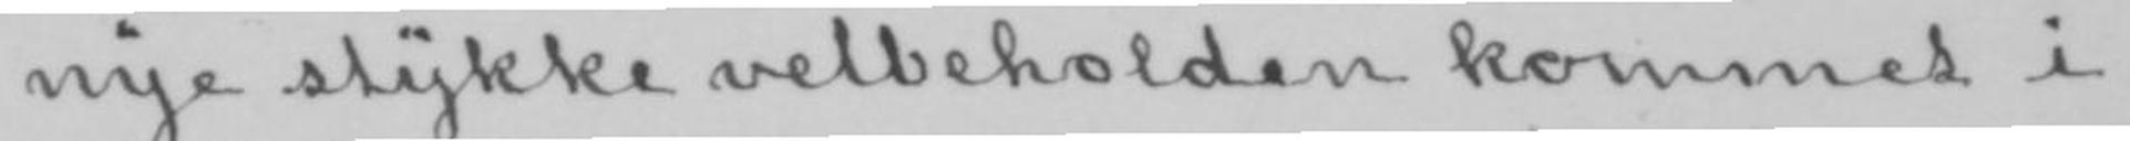nye stykke velbeholden kommet i
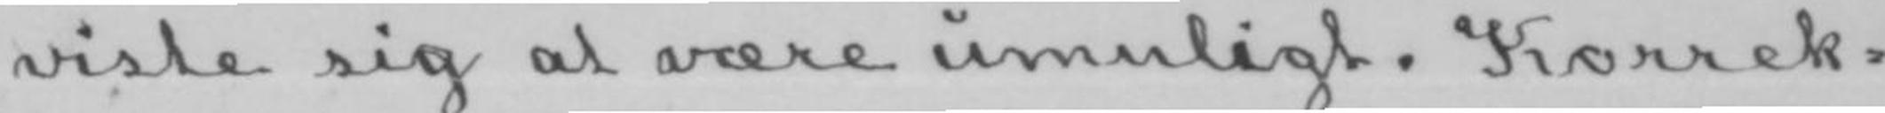viste sig at være umuligt. Korrek-
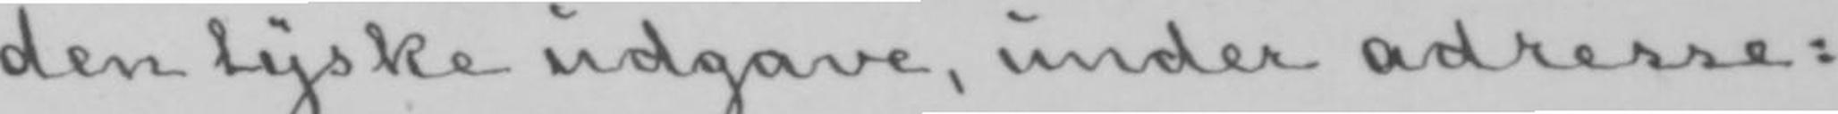den tyske udgave, under adresse:
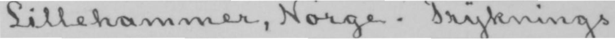Lillehammer, Norge. Tryknings-
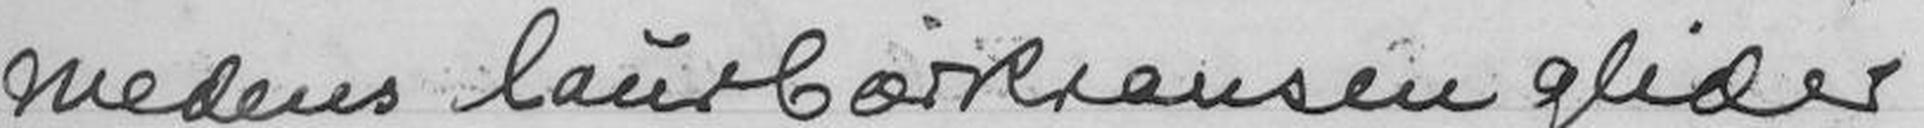medens laurbærkransen glider
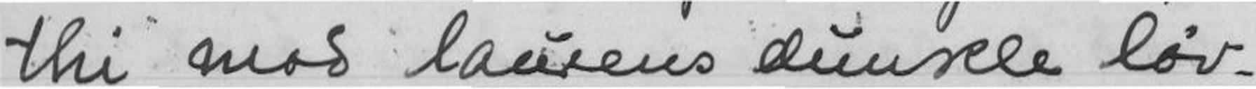thi mod laurens dunkle løv -
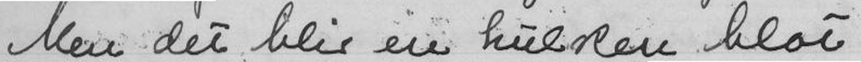Men det blir en hulken blot
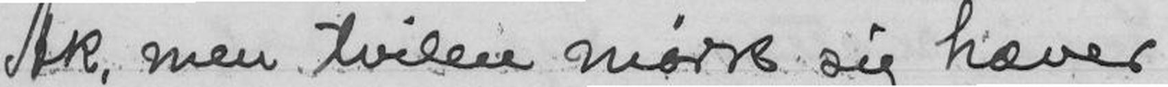Ak, men tvilen mørkt sig hæver -
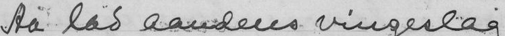Aa, lad aandens vingeslag
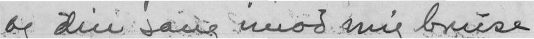og din sang imod mig bruse
